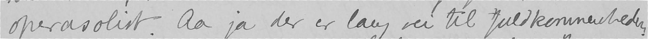operasolist. Aa ja der er lang vei til fuldkommenheden.
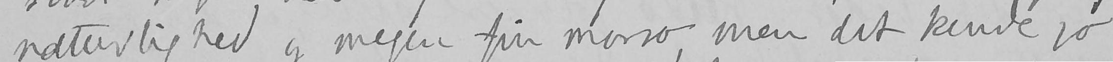naturlighed og megen fin morro, men det kunde jo
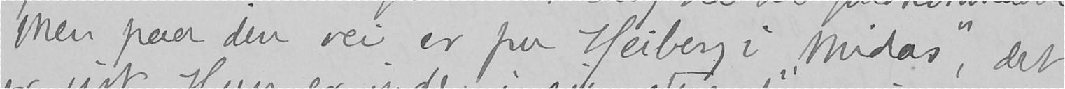Men paa den vei er fru Heiberg i "Midas", det
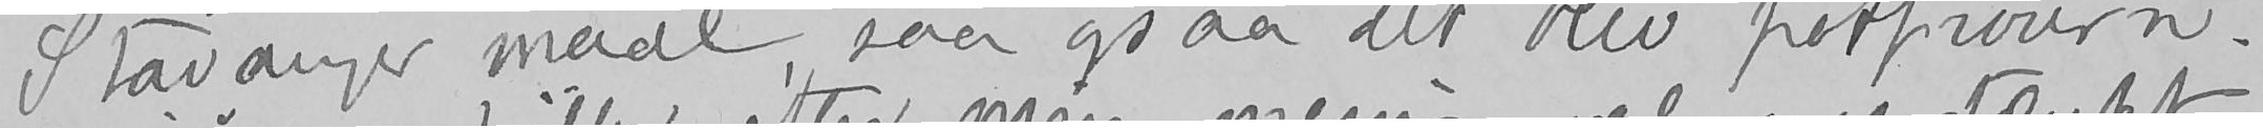Stavanger maal, saa ogsaa det blev potpourri.
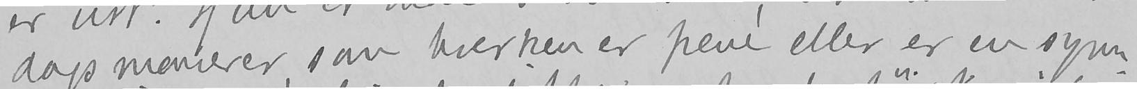dagsmanerer, som hverken er pene eller er en sym-
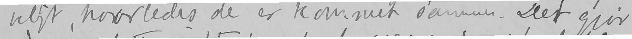beligt, hvorledes de er kommet sammen. Det gjør
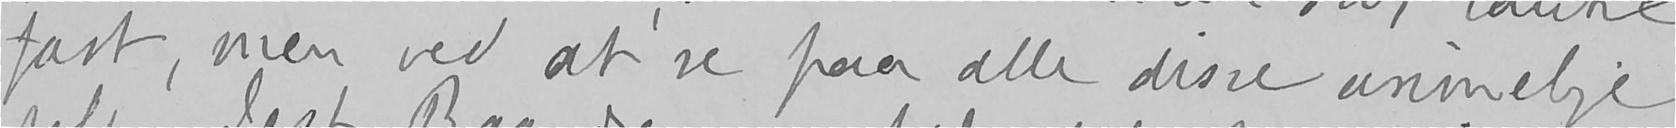fast, men ved at se paa alle disse urimelige
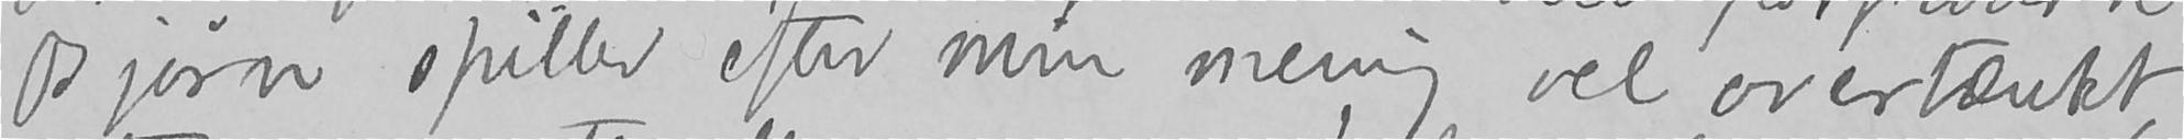Bjørn spiller efter min mening vel overtænkt,
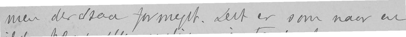men der er saa formeget. Det er som naar en
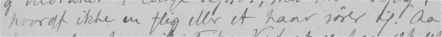hvoraf ikke en flig eller et haar rører sig! Aa
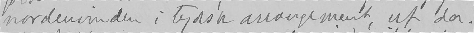nordenvinden i tydsk arrangement, uf da.
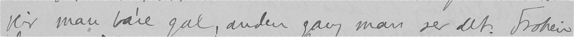blir man bare gal, anden gang man ser det. Frøken
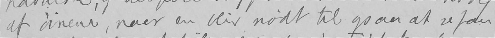af øinene, naar en blir nødt til ogsaa at se paa
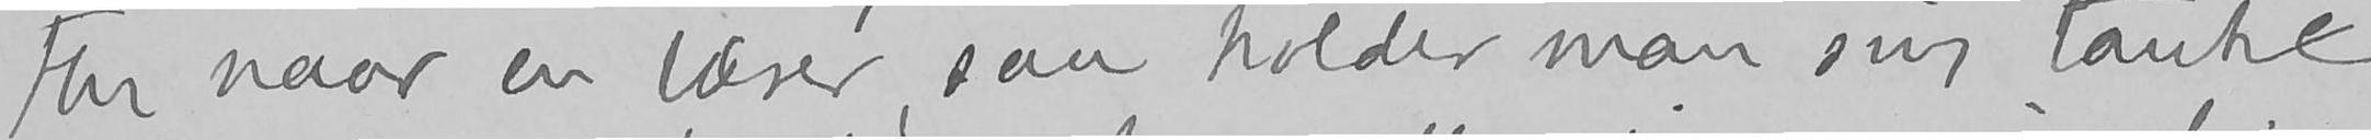for naar en læser, saa holder man sin tanke
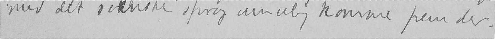med det svænske sprog umulig komme frem der.
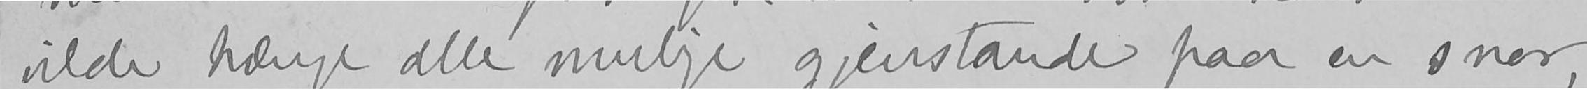vilde hænge alle mulige gjenstande paa en snor,
